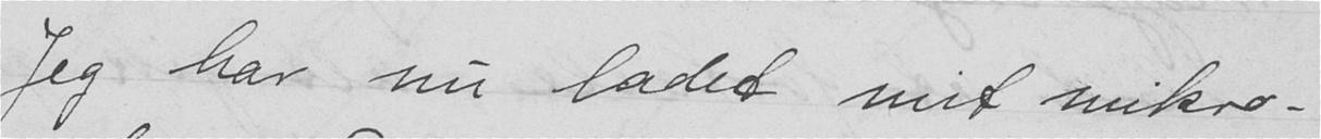Jeg har nu ladet mit mikro-
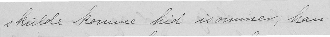skulde komme hid isommer; han
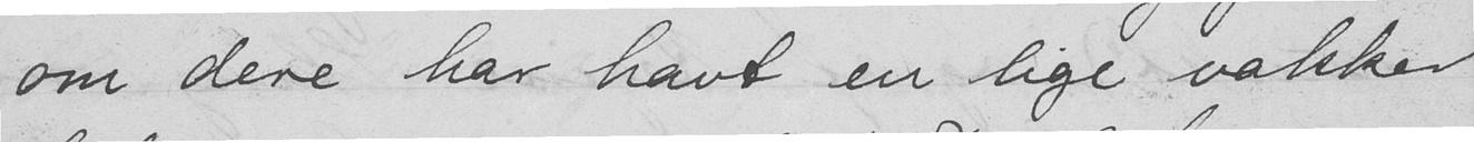om dere har havt en lige vakker
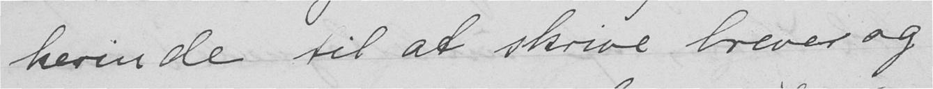herinde til at skrive brever og
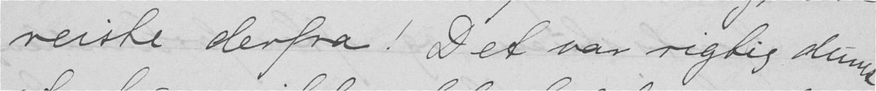reiste derfra! Det var rigtig dumt
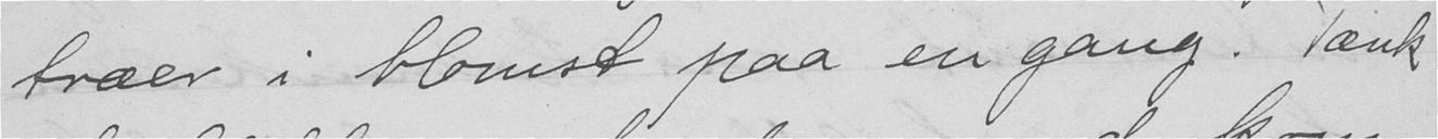træer i blomst paa en gang. Tænk
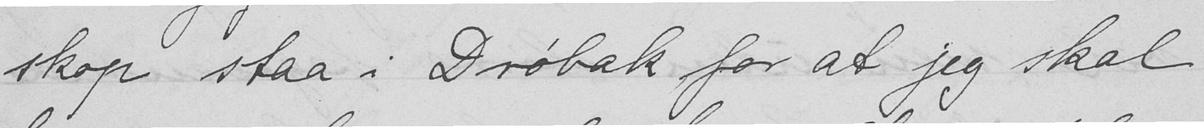skop staa i Drøbak for at jeg skal
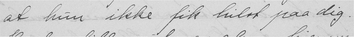at hun ikke fik hilst paa dig.
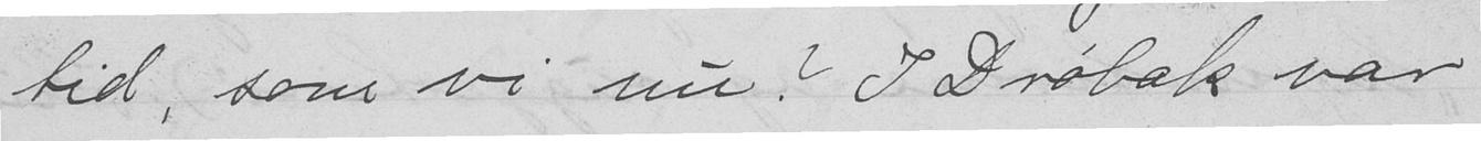tid, som vi nu? I Drøbak var
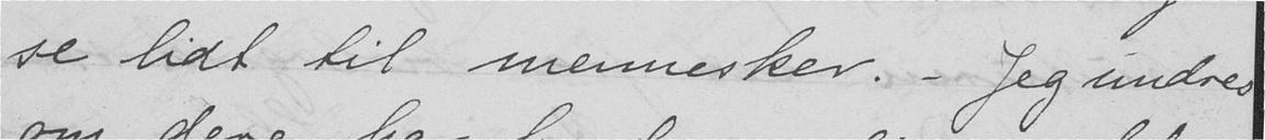se lidt til mennsker. - Jeg undrer
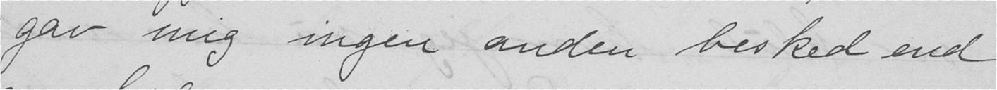gav mig ingen anden besked end
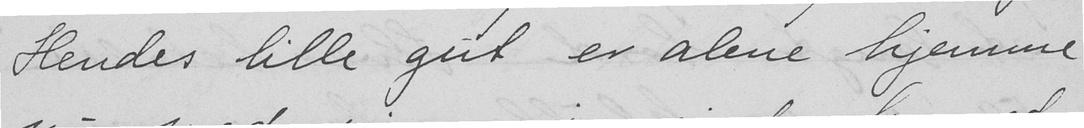Hendes lille gut er alene hjemme
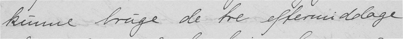kunne bruge de tre eftermiddage
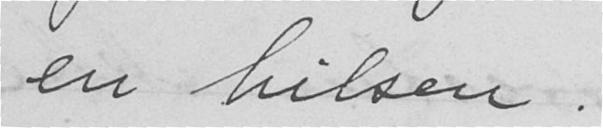en hilsen.
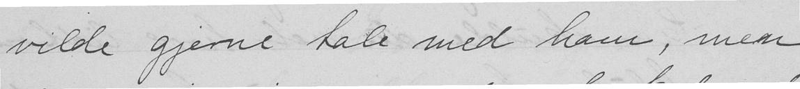vilde gjerne tale med ham, men
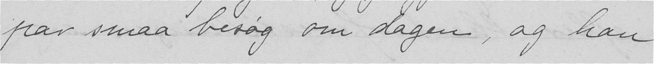par smaa besøg om dagen, og han
